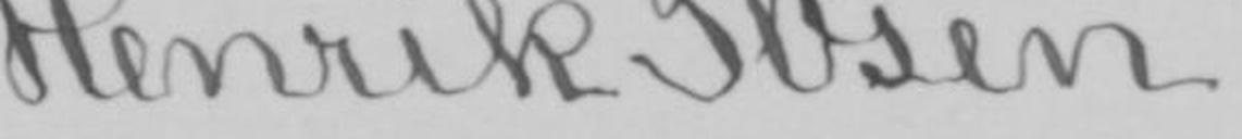Henrik Ibsen.
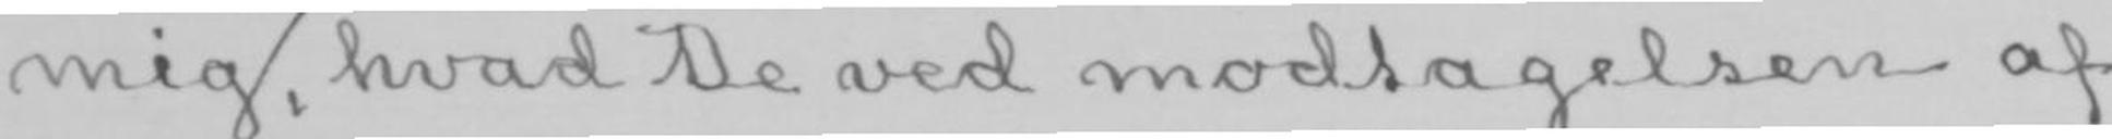mig, hvad De ved modtagelsen af
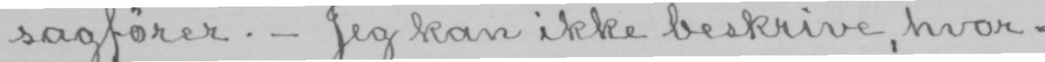sagfører.- Jeg kan ikke beskrive, hvor-
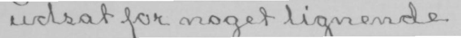udsat for noget lignende.
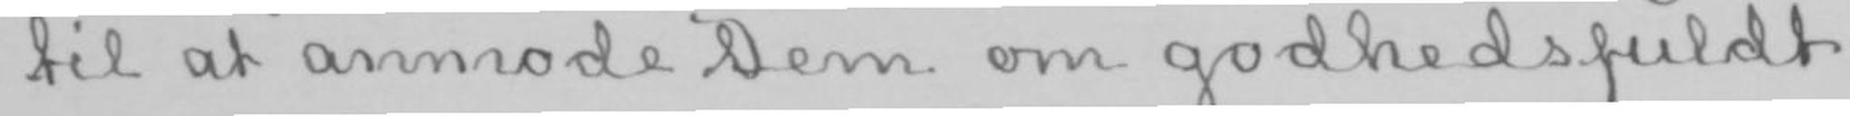til at anmode Dem om godhedsfuldt
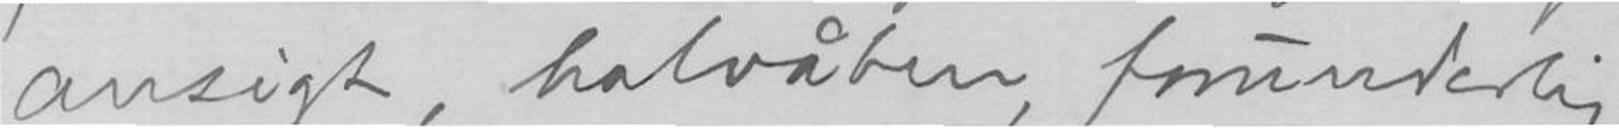ansigt, halvåben, forunderlig
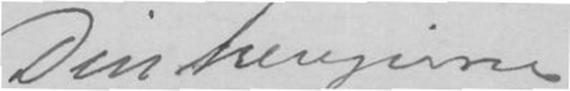Din hengivne
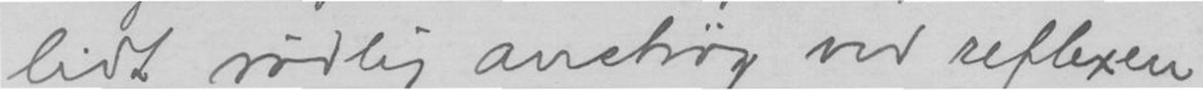lidt rødlig anstrøg ved reflexen
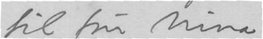til fru Nina.
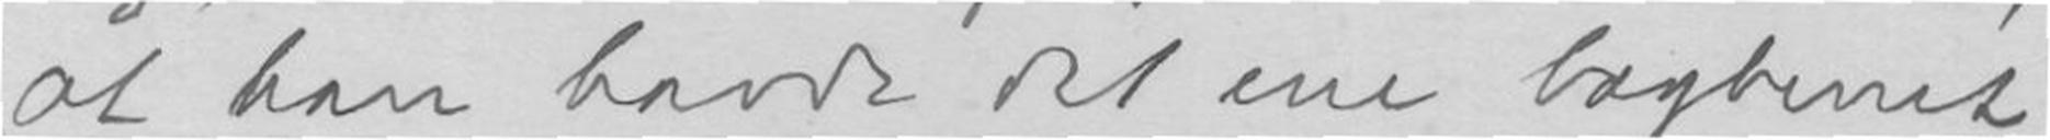at han havde det ene bagbenet
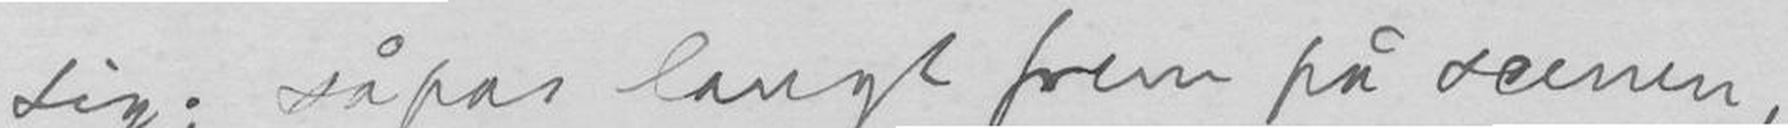sig; såpas langt frem på scenen,
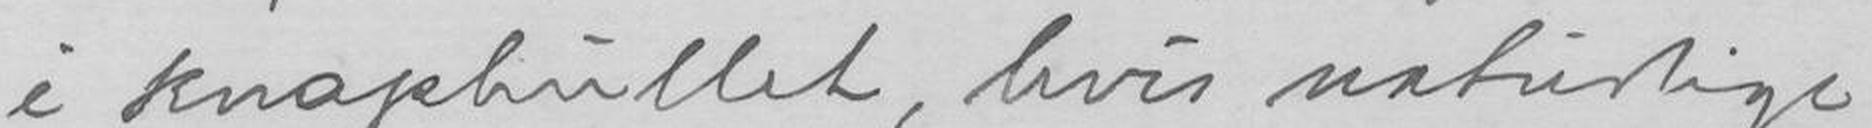i knaphullet, hvis naturlige
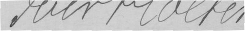Iver Holter
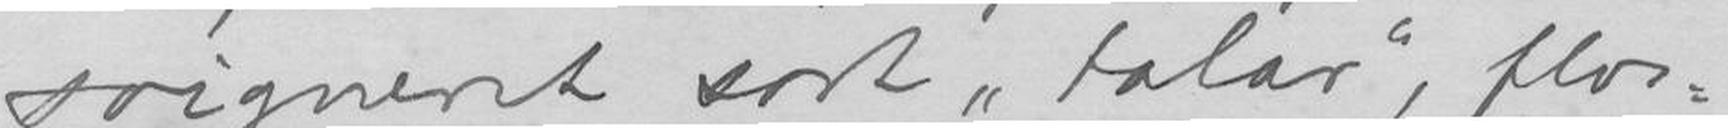soigneret sort "talar", flos-
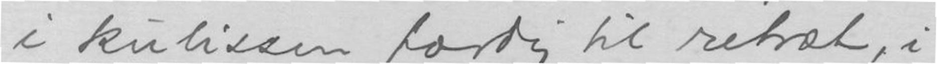i kulissen færdig til retræt, i
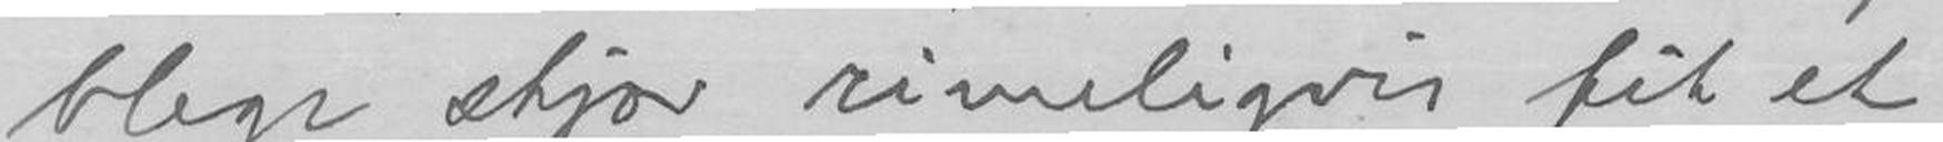blege skjør rimeligvis fik et
